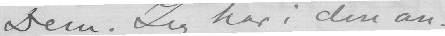Dem. Jeg har i den an-
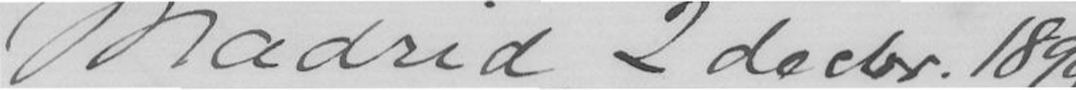Madrid, 2 decbr. 1899
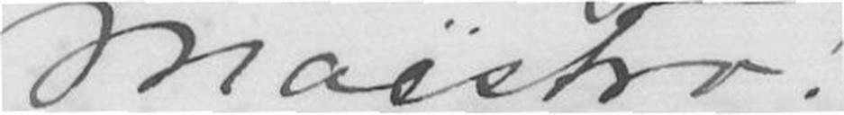Mäestro!
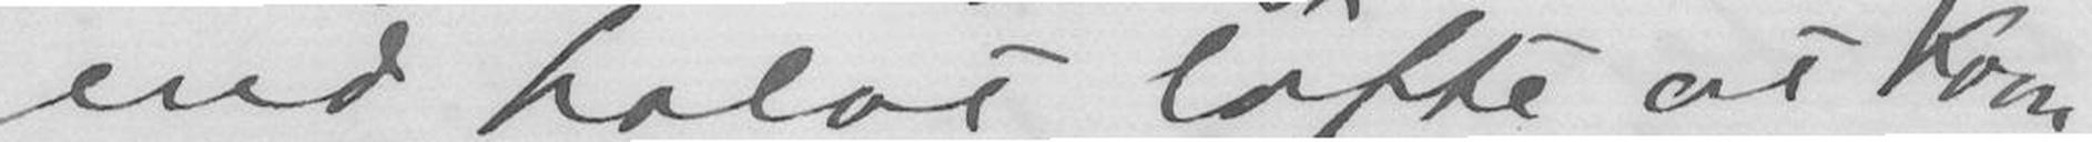end halvt løfte at Kom
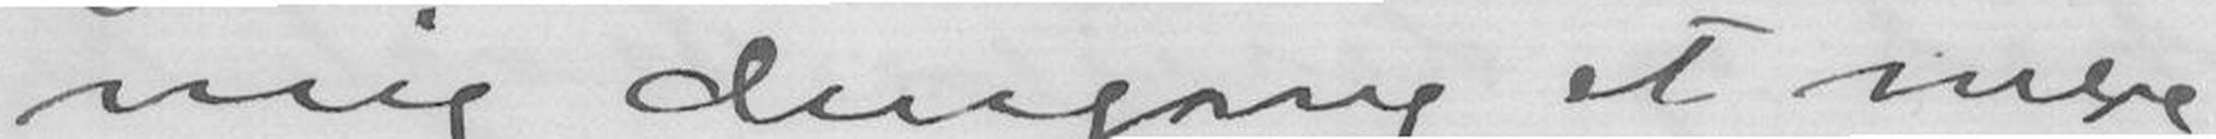mig dengang et mere
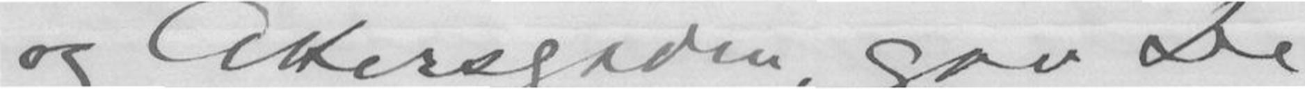og Akersgaden, gav De
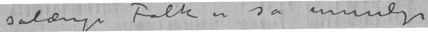sålænge Folk er så umulige
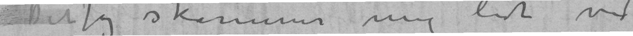Det Jeg skammer mig lidt ved
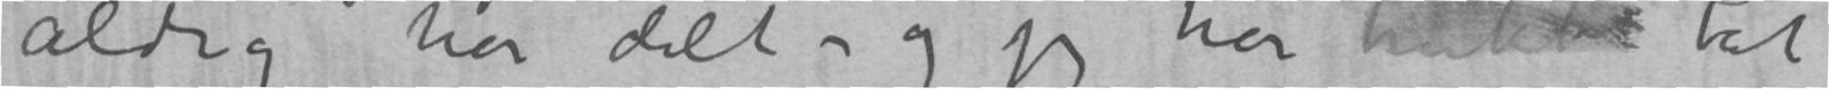aldrig har delt – og jeg har trukket tat
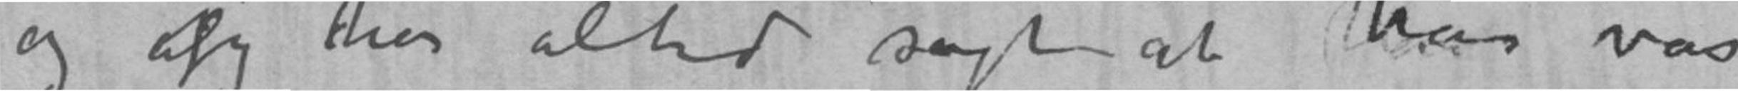og jeg har altid sagt at han var
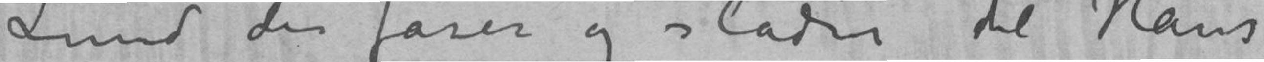Lund der farer og sladrer til Hans
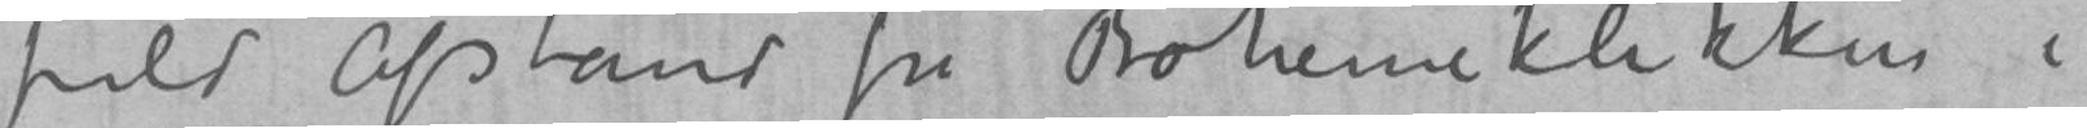fuld Afstand fra Bohemklikken i
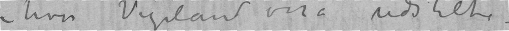– hvor Vigeland osså udstilte –
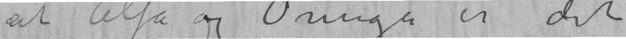at Alfa og Omega er det
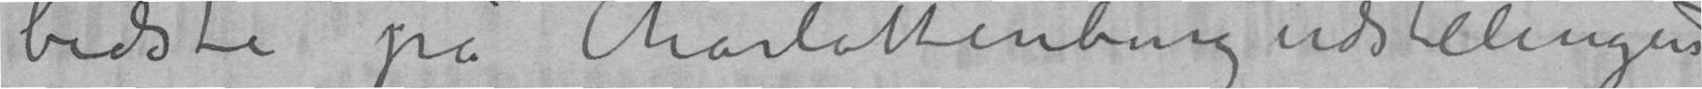bedste på Charlottenburgudstillingen
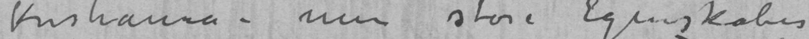Kristiania – men store Egenskaber
